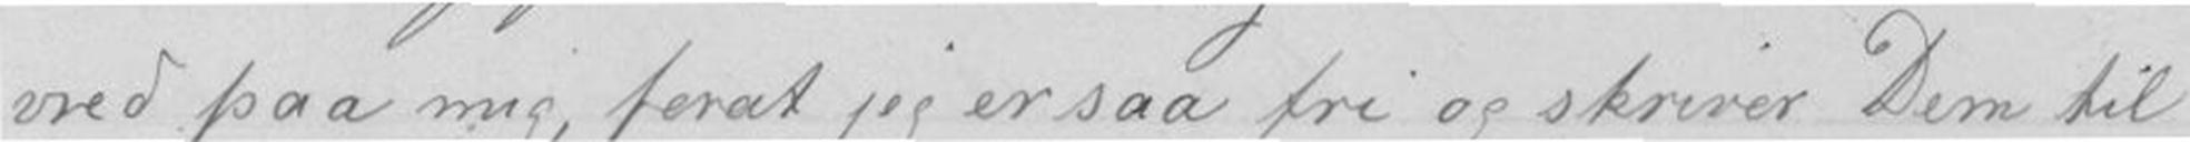vred paa mig, forat jeg er saa fri og skriver Dem til
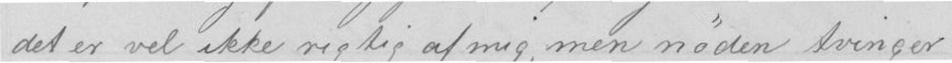det er vel ikke rigtig af mig, men nøden tvinger
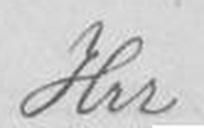Hrr
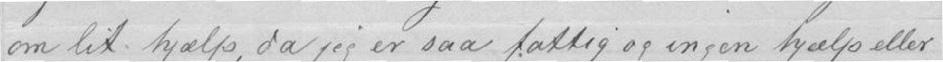om lit hjælp, da jeg er saa fattig og ingen hjælp eller
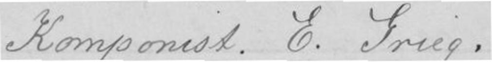Komponist E. Grieg.
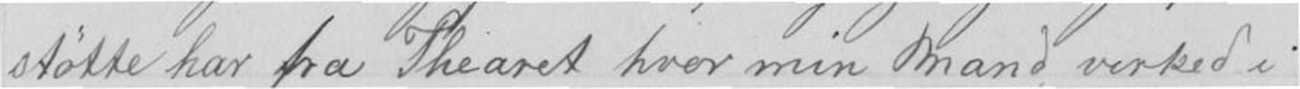støtte har fra Thearet, hvor min Mand virked i
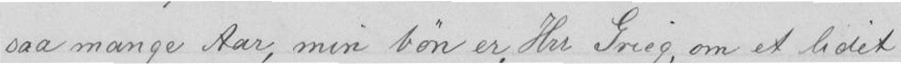saa mange Aar, min bøn er, Hrr Grieg, om et lidet
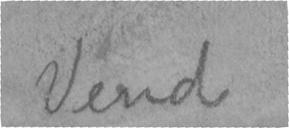Vend
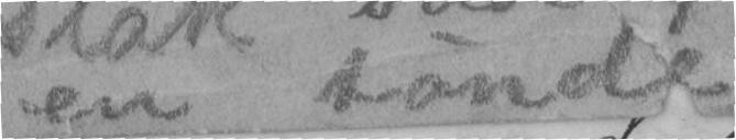en tønde
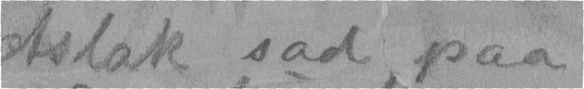Aslak sad paa
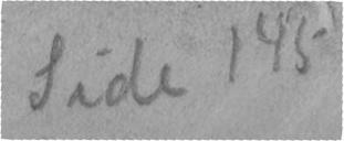Side 143
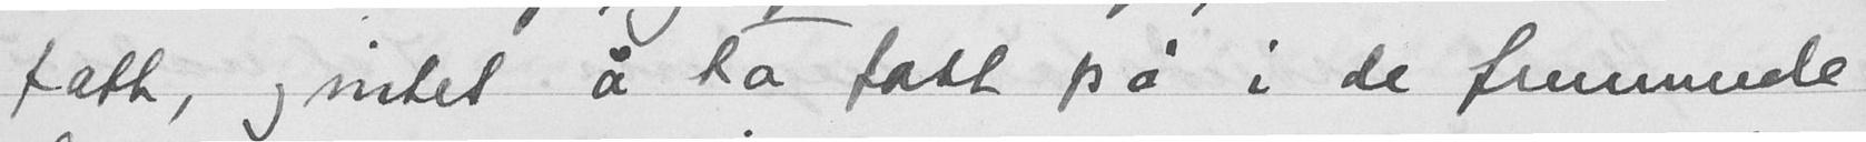fatt, og intet å ta fatt på i de fremmede
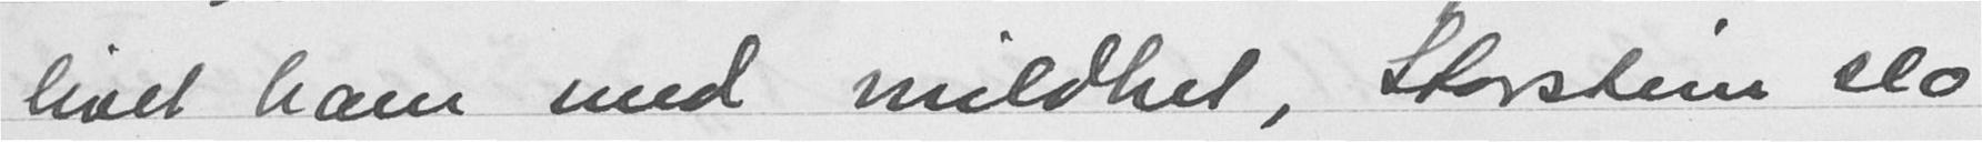livet ham med mildhet, Storstein slo
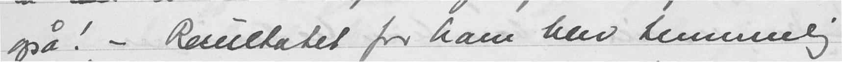også! - Resultatet for ham blev temmelig
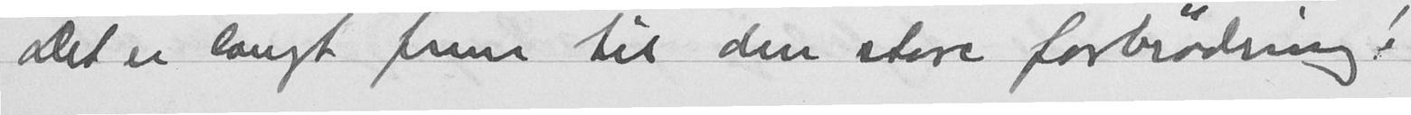Det er langt frem til den store forbrødring.
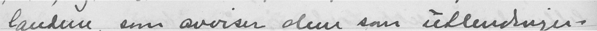landene, som avviser dem som utlendingen
